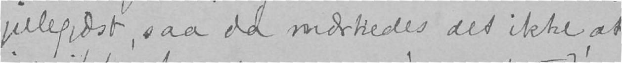julegjæst, saa da mærkedes det ikke, at
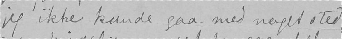jeg ikke kunde gaa med noget sted,
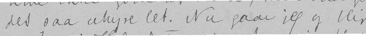det saa uhyre let. Nu gaar jeg og blir
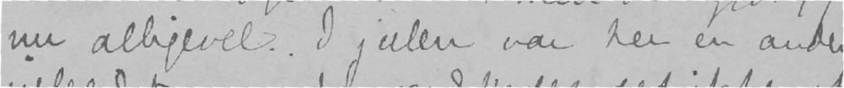nu alligevel. I julen var her en anden
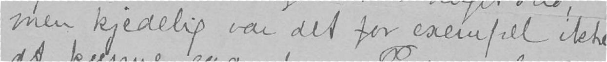men kjedelig var det for exempel ikke
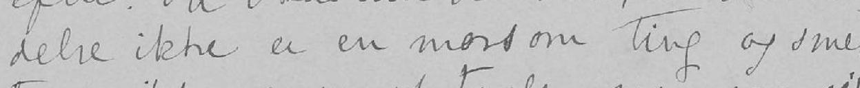delse ikke er en morsom ting og smer-
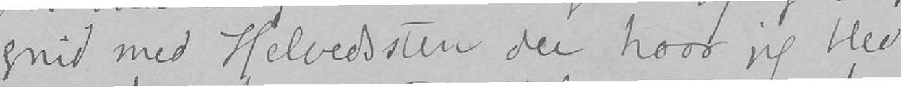gnid med Helvedssten der hvor jeg blev
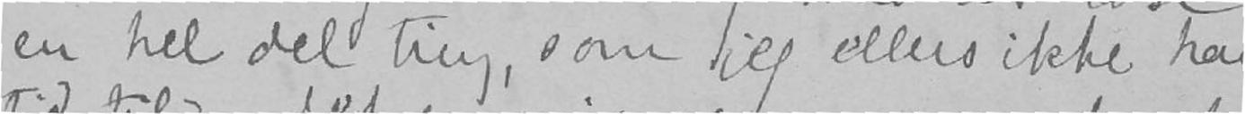en hel del ting, som jeg ellers ikke har
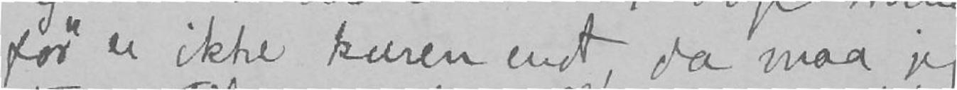før er ikke kuren endt, da maa jeg
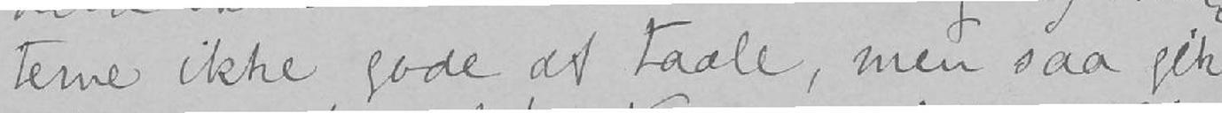terne ikke godt at taale, men saa gik
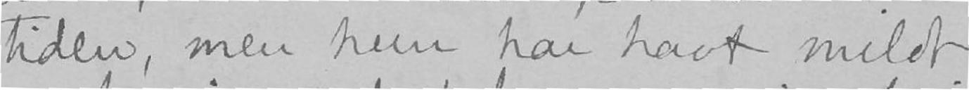tiden, men hun har havt mildt
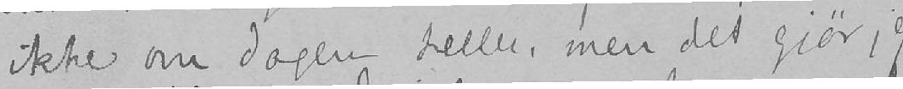ikke om dagen heller, men det gjør jeg
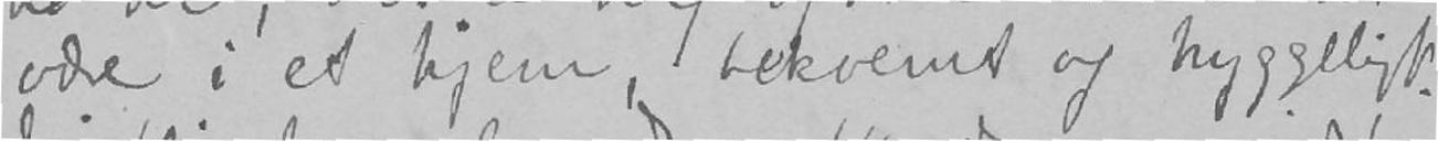være i et hjem, bekvemt og hyggeligt.
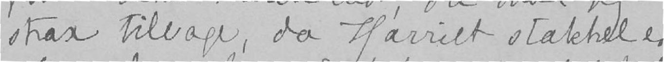strax tilbage, da Harriet stakkel er
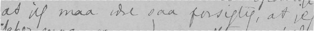at jeg maa være saa forsigtig, at jeg
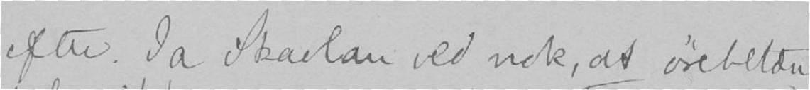efter. Ja Skavlan ved nok, at ørebetæn-
